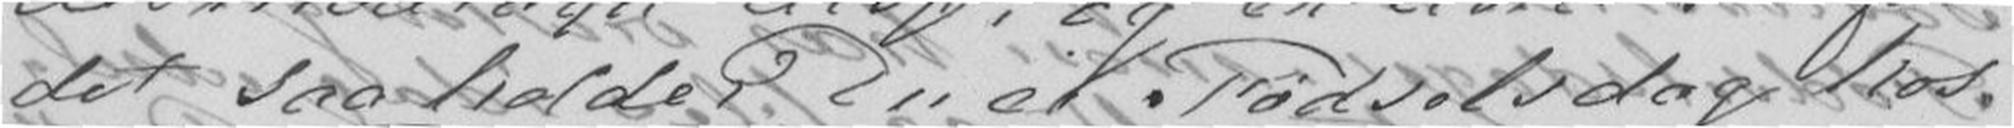det saaholder Du ei Fødselsdag hos
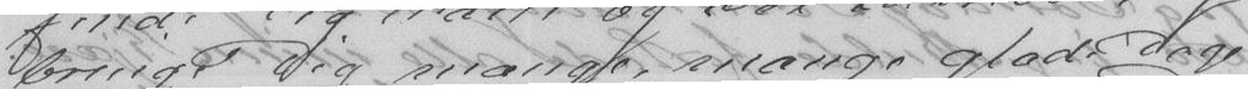bruge Dig mange, mange glade Dage
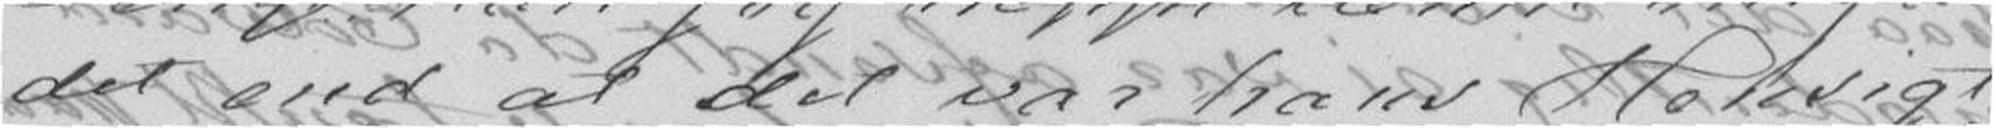det end at det var hans Hensigt
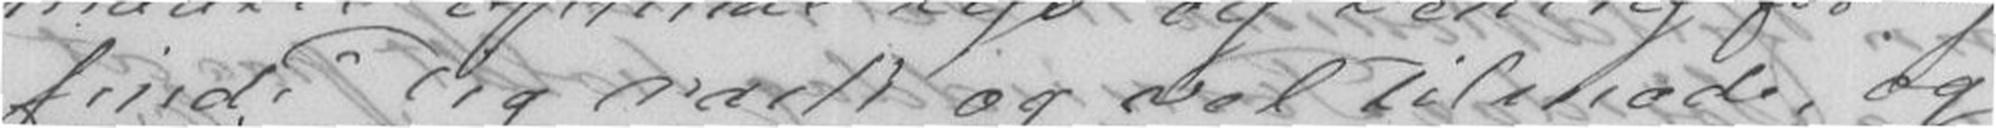finde Dig rask of vel tilmode, og
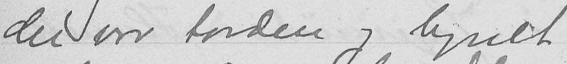der var torden og lynet
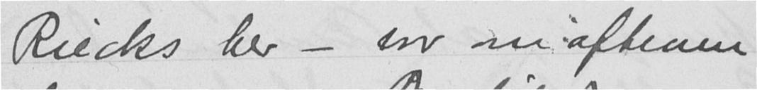Riecks her - var om aftenen
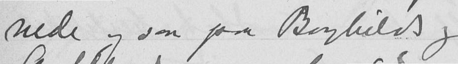nede og saa paa Borghilds og
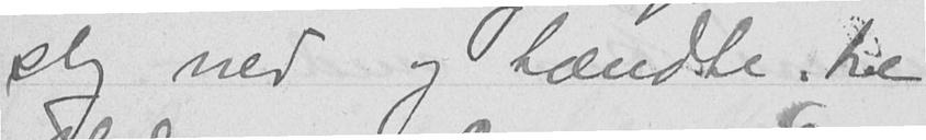slog ned og tændte til
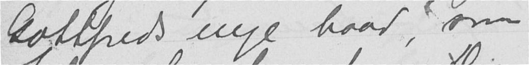Gottfreds nye baad, som
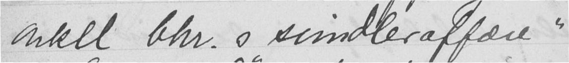onkel Chr.s svindleraffære
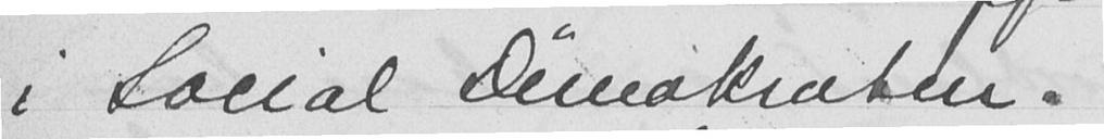i Social Demokraten.
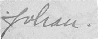Johan.
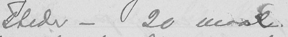steder - 20 maal
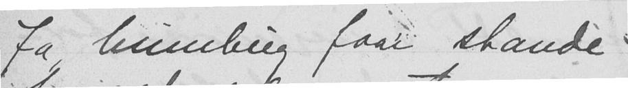Ja, humbug faar stande
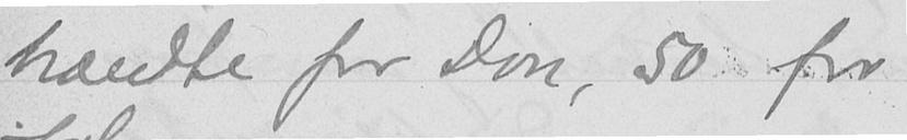brændte for Don, 50 for
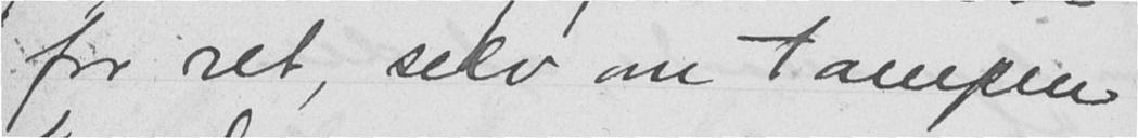for ret, selv om tampen
